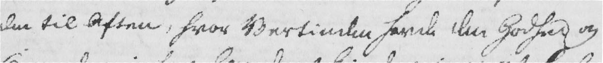den til Aften, hvor Vertinden havde den Godhed og
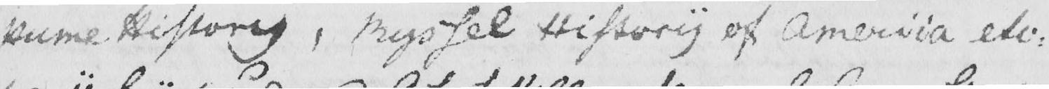Hume History, Myssel History of America etc.
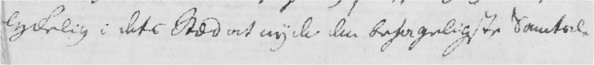lyckelig i dets Stæd at nyde den behageligste Samtale
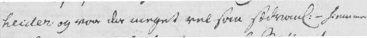heider og var der meget vel som sædvanl. - hiemme
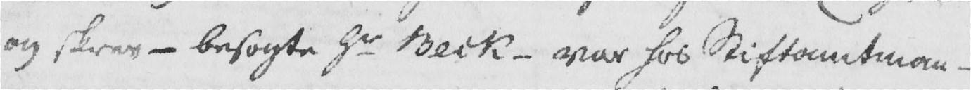og skrev - besøgte Hr Beck - var hos Stiftamtman-
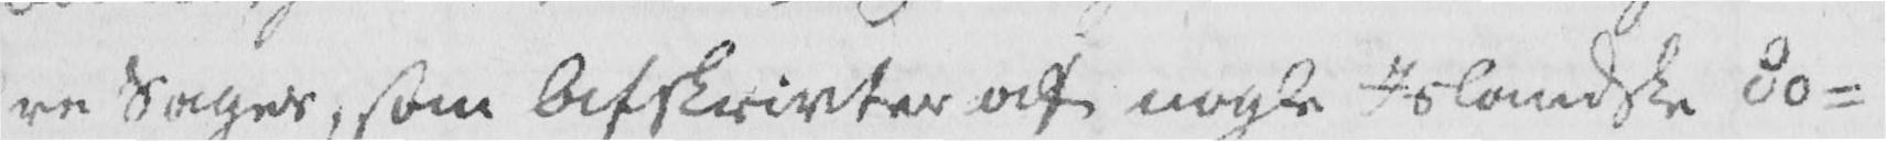re Sager, som Afskrivter af nogle Islandske Co-
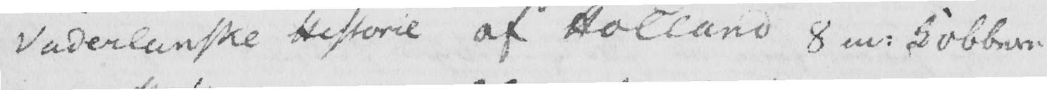Vaderlanske Historie af Holland 8 m. Kobbere,
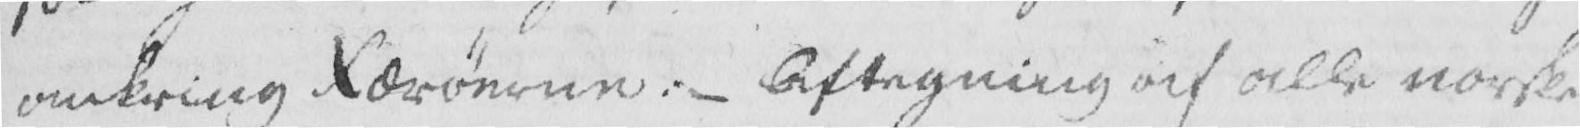omkring Færøerne. - Aftegning af alle norske
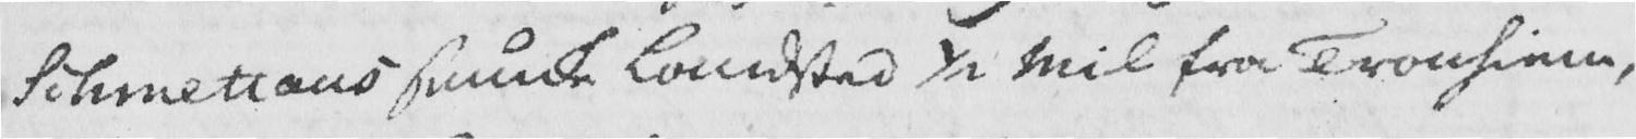Schmettaus smucke Landsted 1/2 Mil fra Tronhiem,
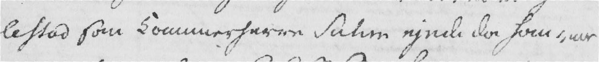lestad som Kammerherre Suhm ejede da han var
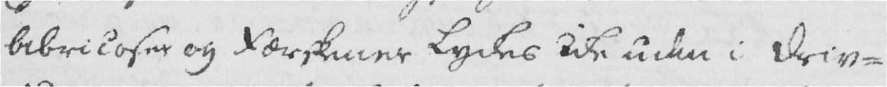Abricoser og Færskener Lyckes icke uden i Driv-
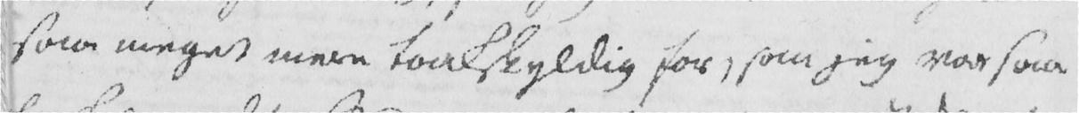saa meget mere tackskyldig for, som jeg var saa
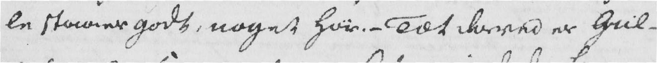le staaer godt, noget Høi. - Tæt derved er Gril-
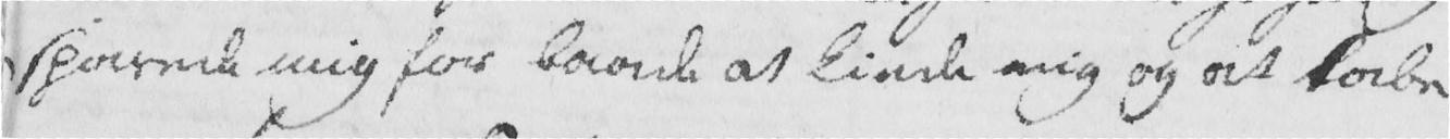sparede mig for baade at kiede mig og at tabe
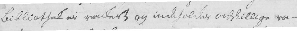Bibliothek er vackert og indeholder adskillige ra-
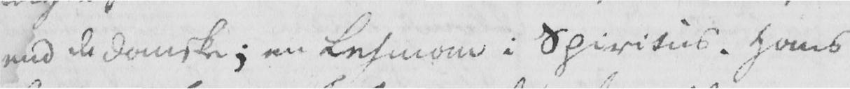end de danske; en Lehmon i Spiritus. Hans
